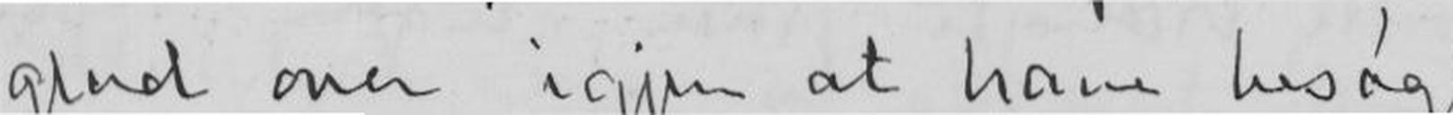glad over igjen at have besøgt
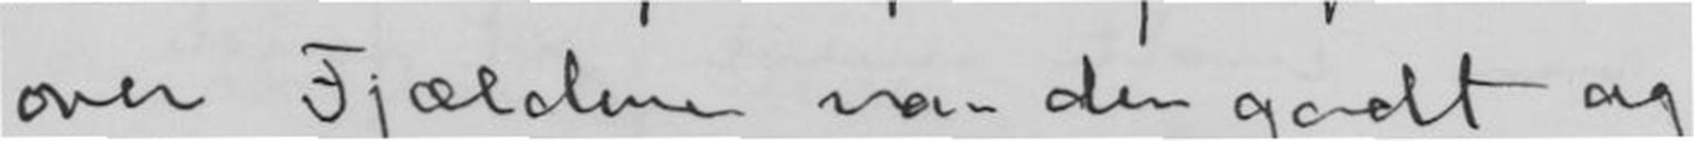over Fjældene var der godt og
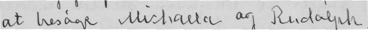at besøge Michelle og Rudolph.
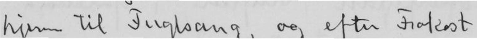hjem til Fuglsang, og efter Frokost
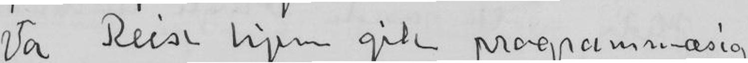Vor Reise hjem gik programmæsig,
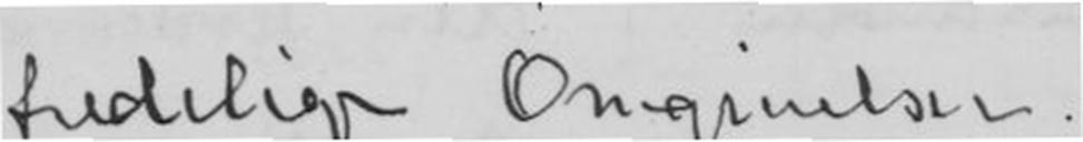fredelige Omgivelser.
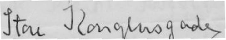Store Kongensgade
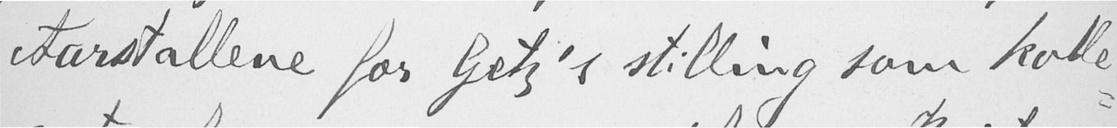Aarstallene for Getz's stilling som kolle-
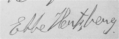Ebbe Hertzberg.
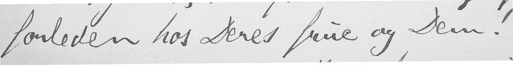forleden hos Deres frue og Dem!
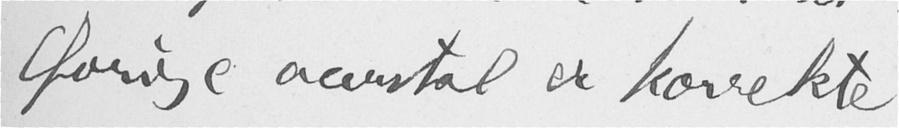Øvrige aarstal er korrekte.
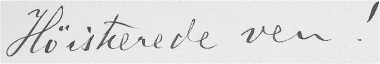Høistærede ven!
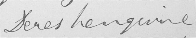Deres hengivne
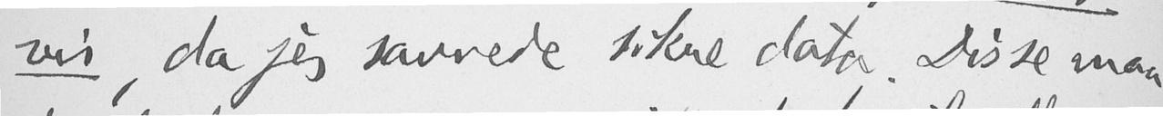vis, da jeg savnede sikre data. Disse maa
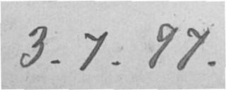3. 7. 97.
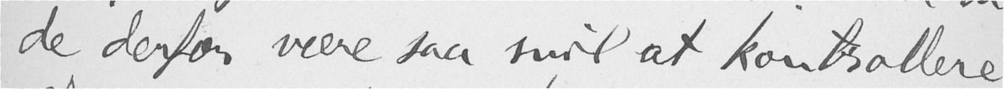de derfor være saa snil at kontrollere.
